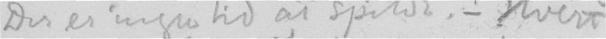Der er ingen tid at spilde. - Hvert
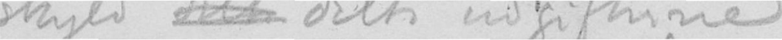skyld delt delte udgifterne
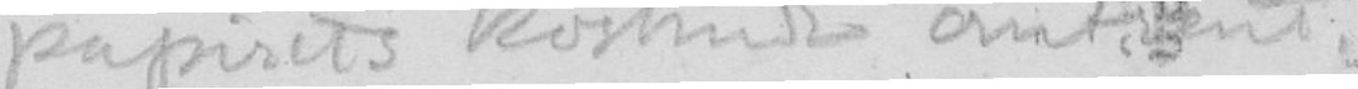papirets kostende omtrent.
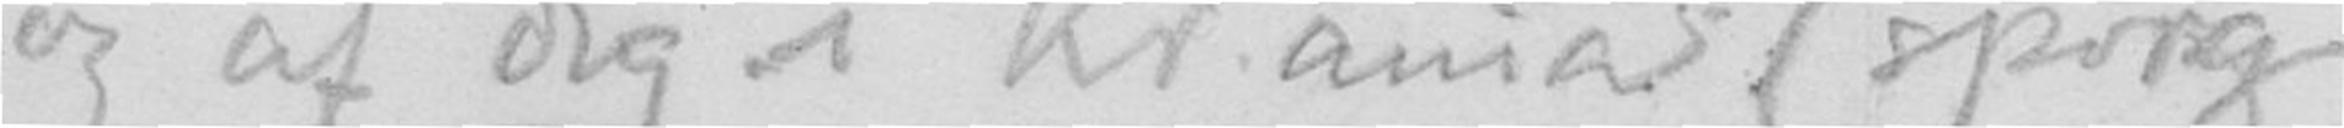og af dig i Kr.ania (spørg
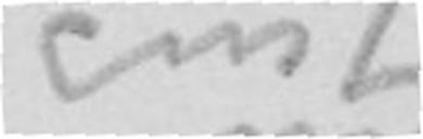Jens
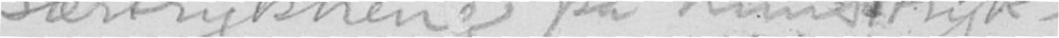særtrykkene på kunsttryk-
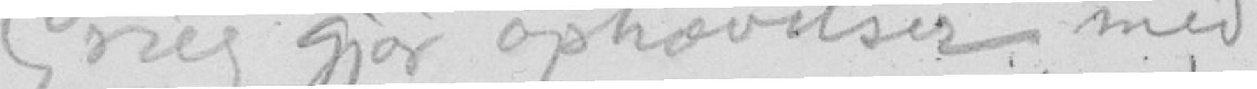Grieg gjør ophævelser med
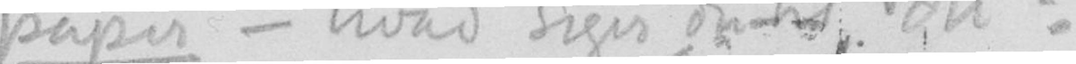papir - hvad siger du til det?
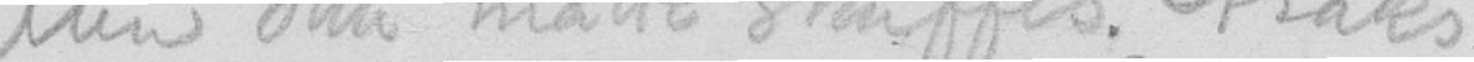Men dette måtte skaffes straks
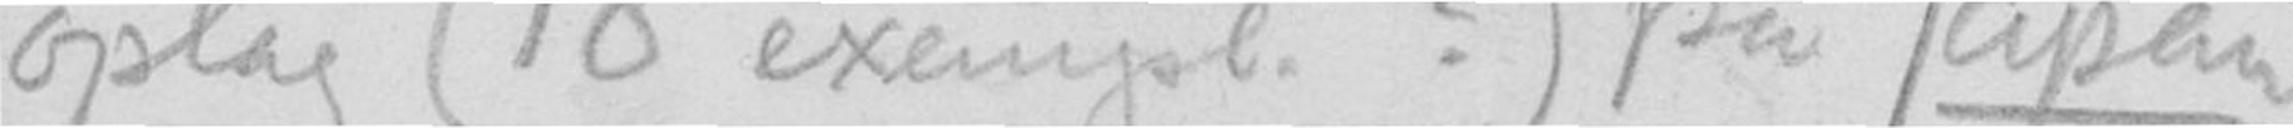oplag (10 exempl.?) på japan-
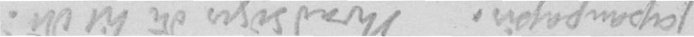japanpapir. Hvad siger du til det?
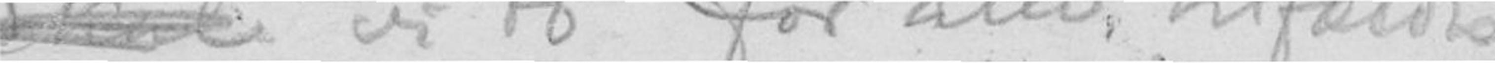Skal vi to for alle tilfældes
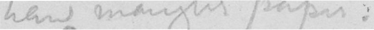han mangler papir.
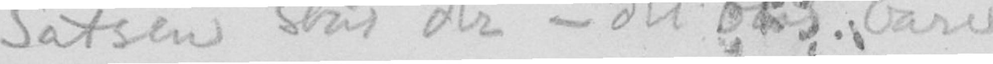Satsen står der - det blir bare
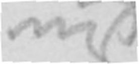Din
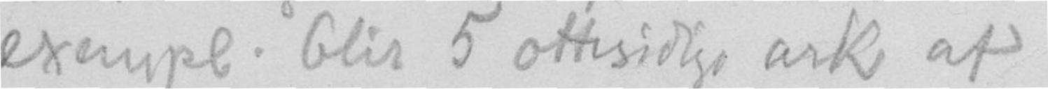exempl. blir 5 ottesidige ark af
